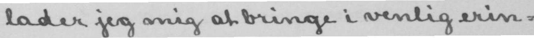lader jeg mig at bringe i venlig erin-
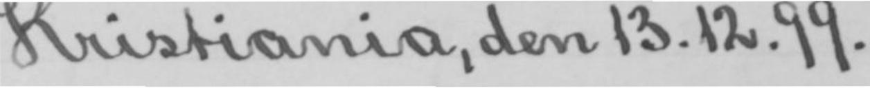Kristiania, den 13.12.99.
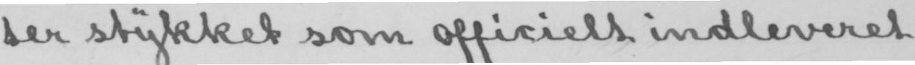ter stykket som officielt indleveret
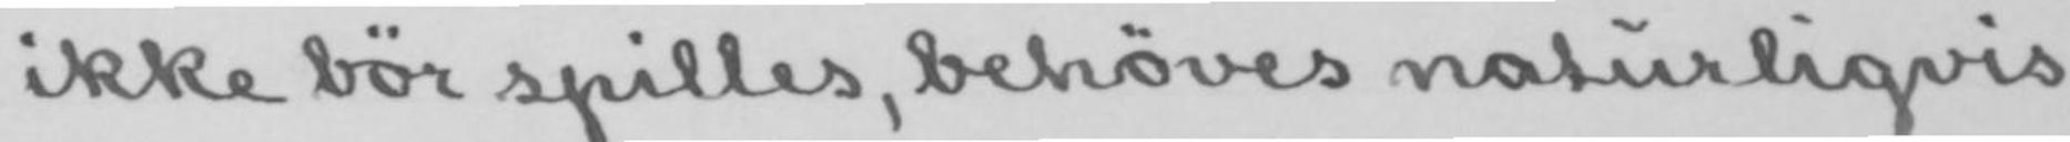ikke bør spilles, behøves naturligvis
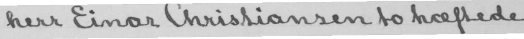herr Einar Christiansen to hæftede
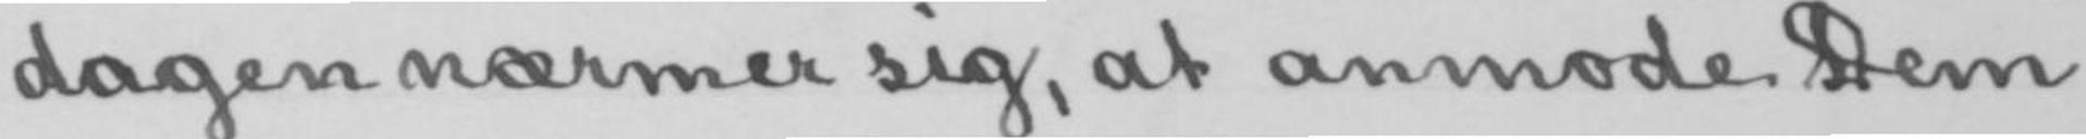dagen nærmer sig, at anmode Dem
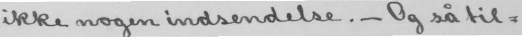ikke nogen indsendelse.- Og så til-
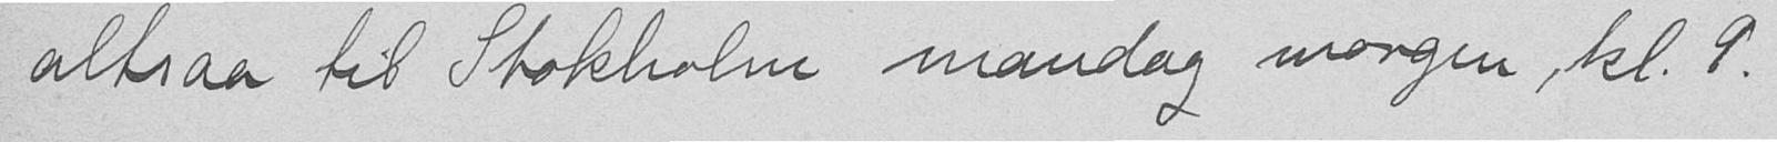altsaa til Stokholm mandag morgen, kl. 9.
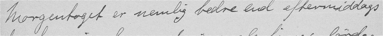Morgentoget er nemlig bedre end eftermiddags-
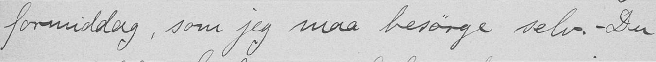formiddag, som jeg maa besørge selv. - Du
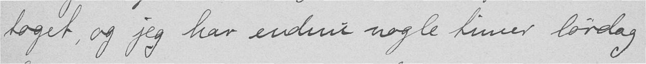toget, og jeg har endnu nogle timer lørdag
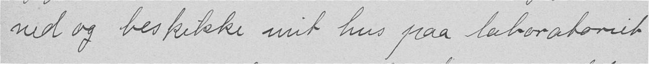ned og beskikke mit hus paa laboratoriet
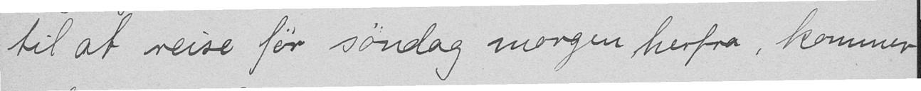til at reise før søndag morgen herfra, kommer
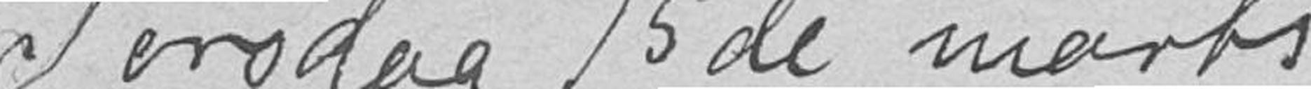Torsdag 15de marts
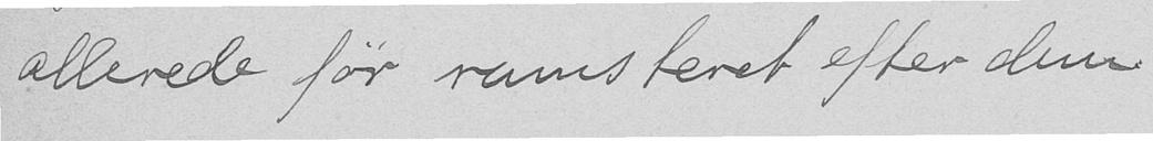allerede før rumsteret efter dem.
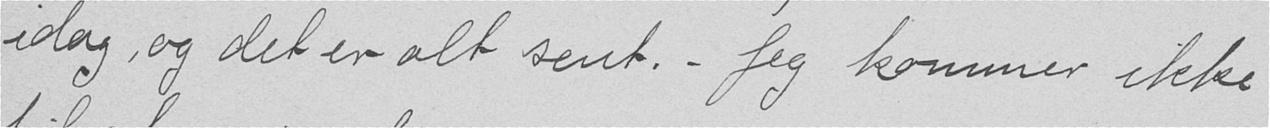idag, og det er alt sent. - Jeg kommer ikke
